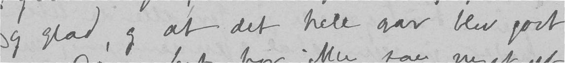og glad, og at det hele aar blev godt
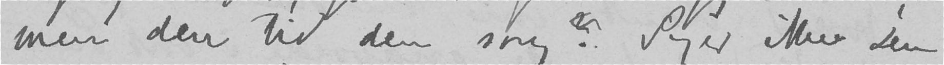men den tid den sorg? Siger ikke Du
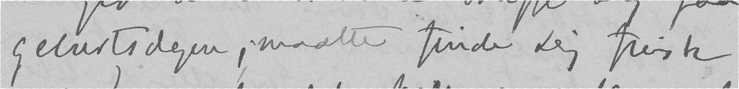geburtsdagen, maatte finde dig frisk
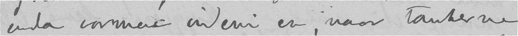enda varmene indeni en, naar tankerne
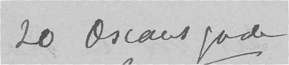20 Oscars gade
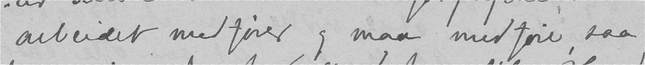arbeidet medfører og maa medføre, saa
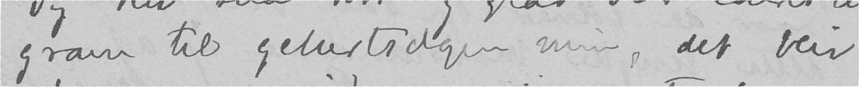gram til geburtsdagen min, det blir
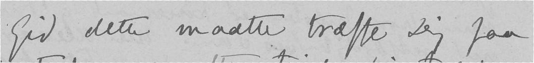Gid dette maatte træffe Dig paa
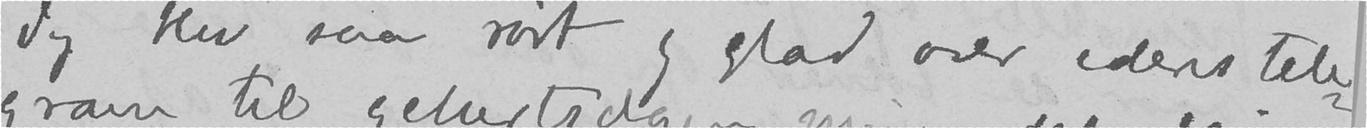Jeg blev saa rørt og glad over eders tele-
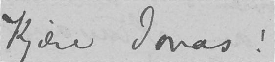Kjære Jonas!
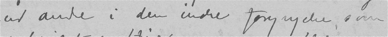end andre i den indre foryngelse, som
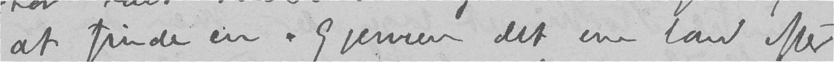at finde en. Gjennem det ene land efter
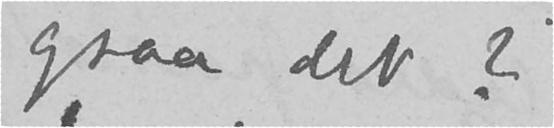ogsaa det?
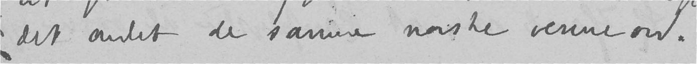det andet de samme norske venneord.
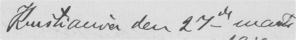Kristiania den 27de marts
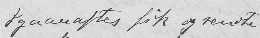Igaaraftes fik og sendte
